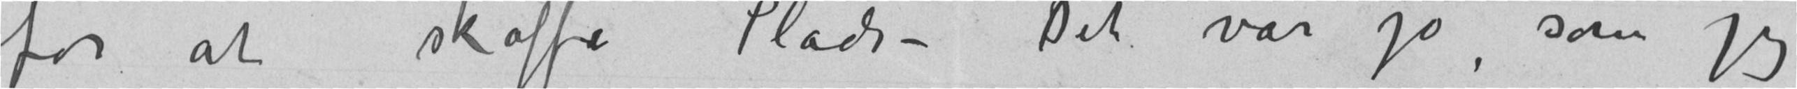for at skaffe Plads – Det var jo som jeg
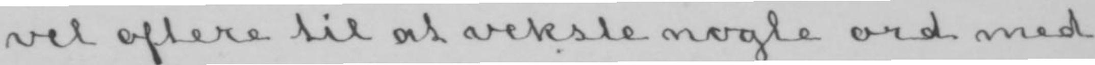vel oftere til at veksle nogle ord med
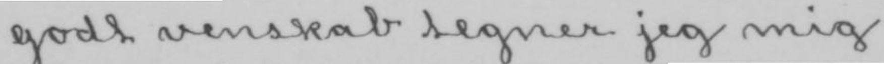godt venskab tegner jeg mig
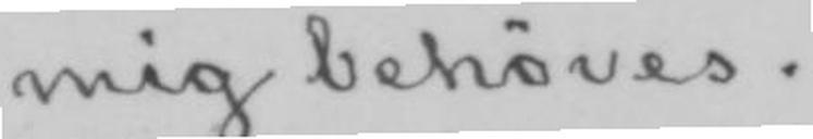mig behøves.
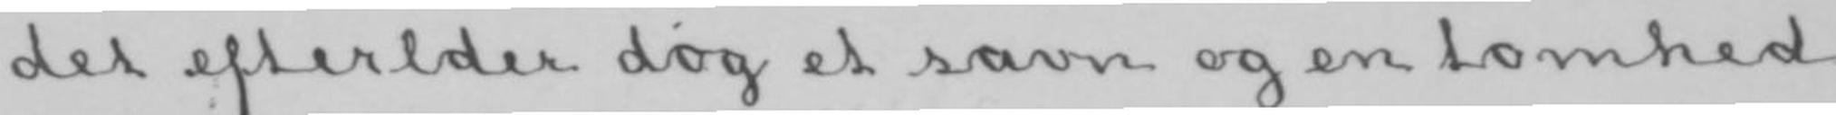det efterlder dog et savn og en tomhed
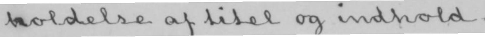holdelse af titel og indhold.
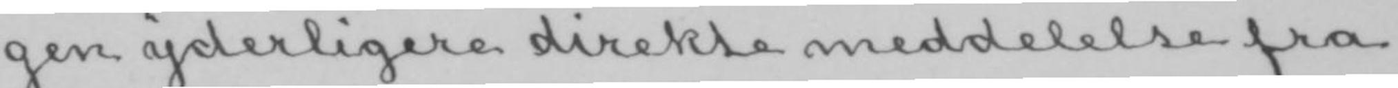gen yderligere direkte meddelelse fra
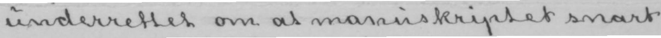underrettet om at manuskriptet snart
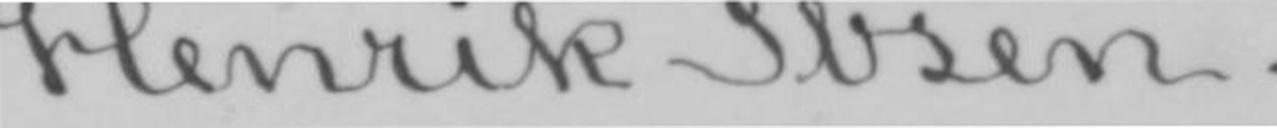Henrik Ibsen.
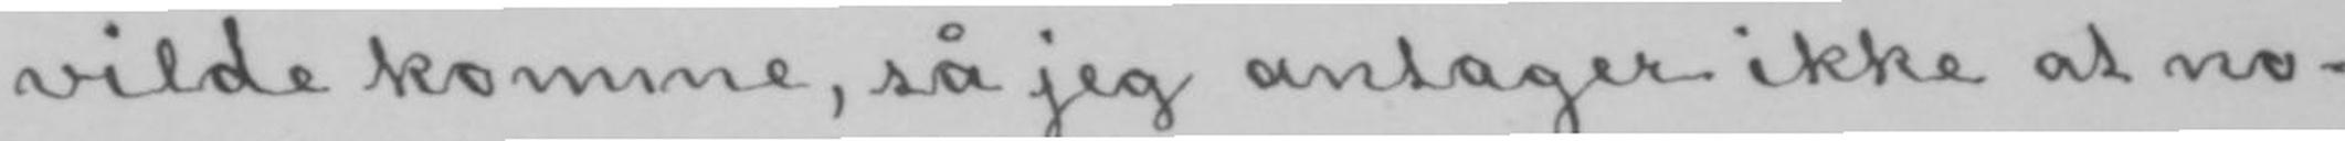vilde komme, så jeg antager ikke at no-
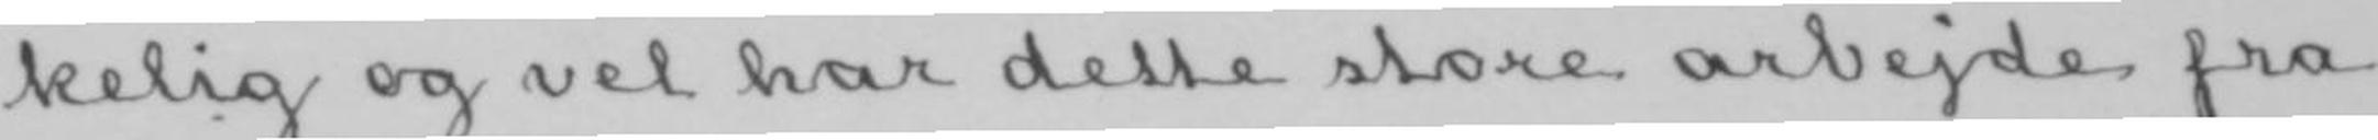kelig og vel har dette store arbejde fra
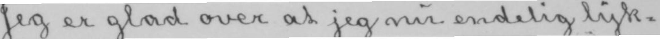Jeg er glad over at jeg nu endelig lyk-
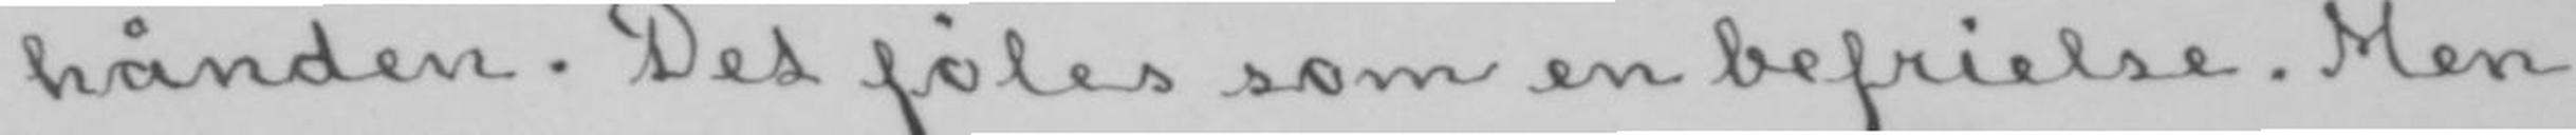hånden. Det føles som en befrielse. Men
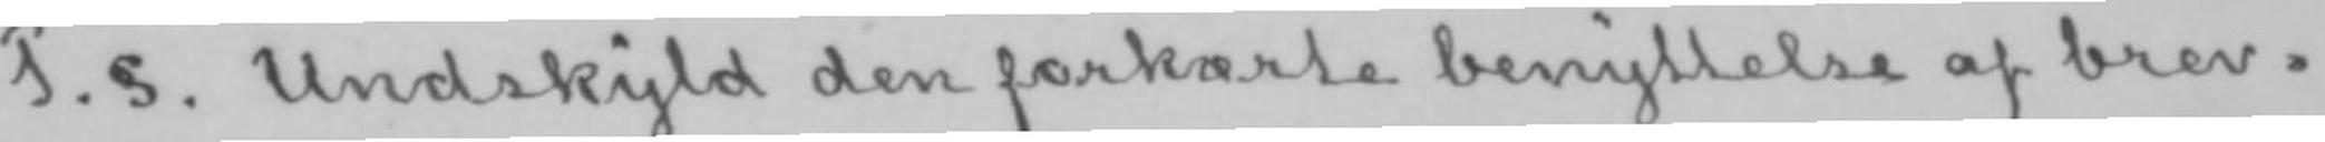P. s. Undskyld den forkærte benyttelse af brev-
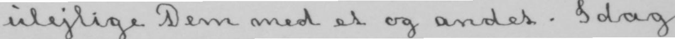ulejlige Dem med et og andet. Idag
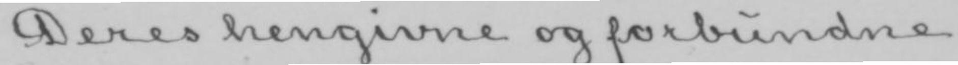Deres hengivne og forbundne
